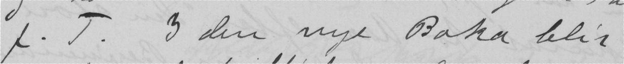f. T. I den nye Boka blir
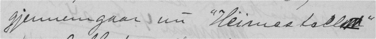gjennemgaar nu "Heimestell"
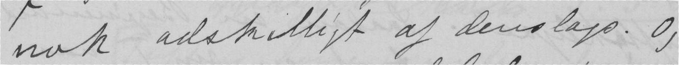nok adskilligt af denslags. Og
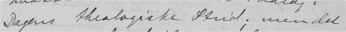Dagens thealogiske Strid; men det
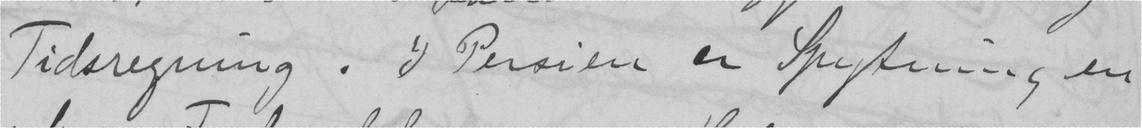Tidsregning. I Persien er Spytning en
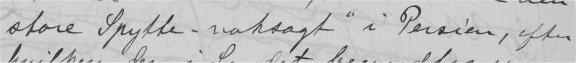store Spytte-noksagt" i Persien, efter
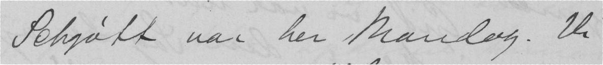Schjøtt var her Mandag. Vi
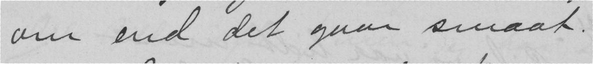om end det gaar smaat.
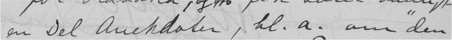en Del Anekdoter, bl. a. om "den
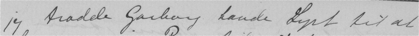jeg trodde Garborg havde Lyst til at
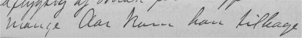mange aar kom han tilbage
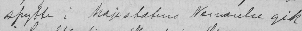spytte i Majestætens Nærværelse gik
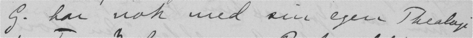G. har nok med sin egen Thealogi
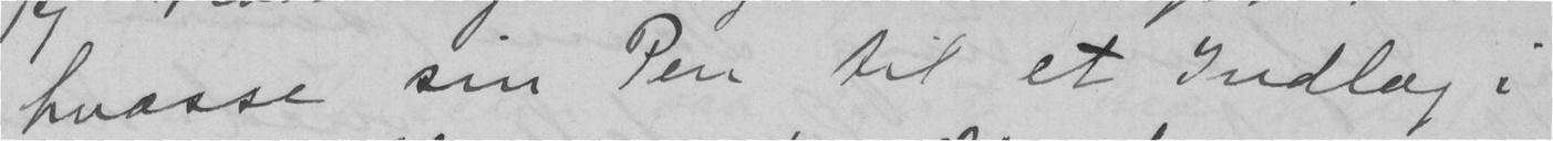hvasse sin Pen til et Indlæg i
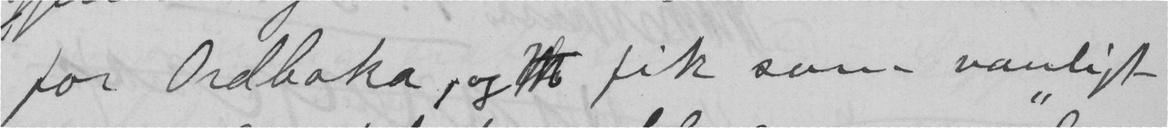for Ordboka, og  fik som vanligt
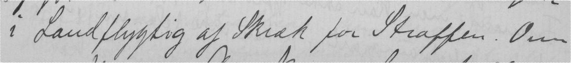i Landflygtig af Skræk for Straffen. Om
